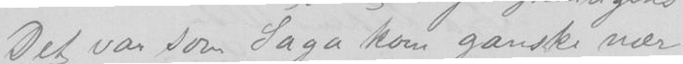Det var som Saga kom ganske nær,
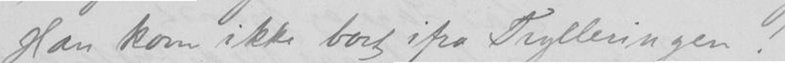Han kom ikke bort ifra Trylleringen!
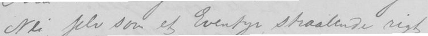Nei, selv som et Eventyr, straalende rigt
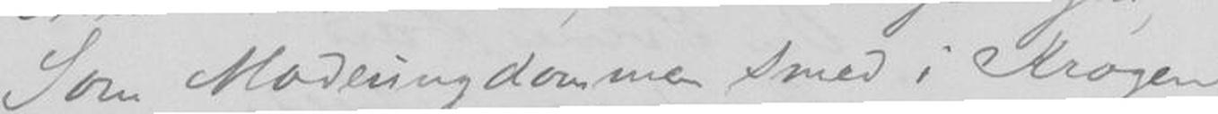Som Modeungdommen smed i Krogen
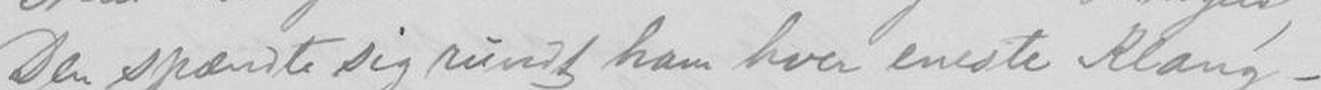Der spændte sig rundt ham hver eneste Klang -
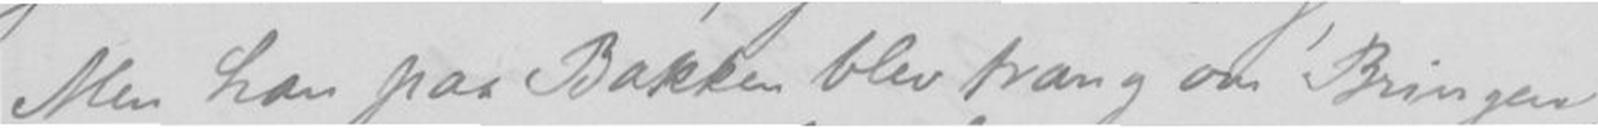Men han paa Bakken blev trang om Bringen,
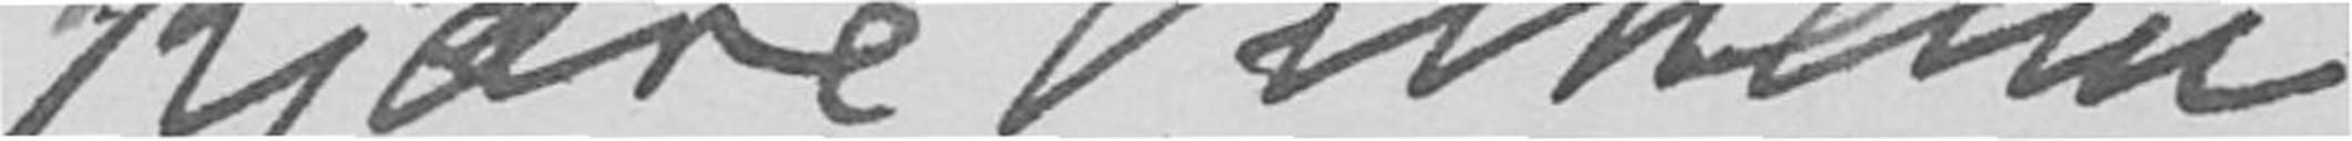Kjære Vilhelm
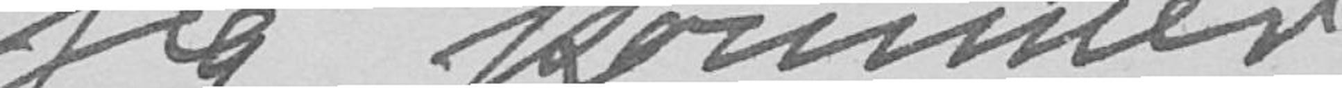jeg kommer
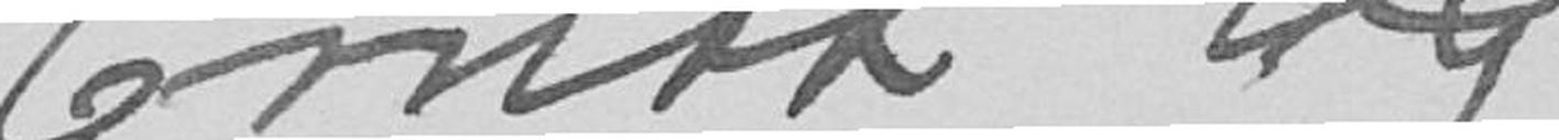Ernst og
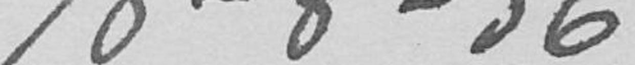18-8-36
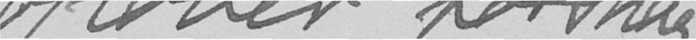opover torsdag
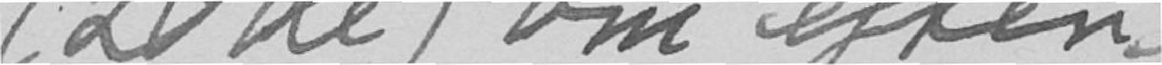(20de) om efter-
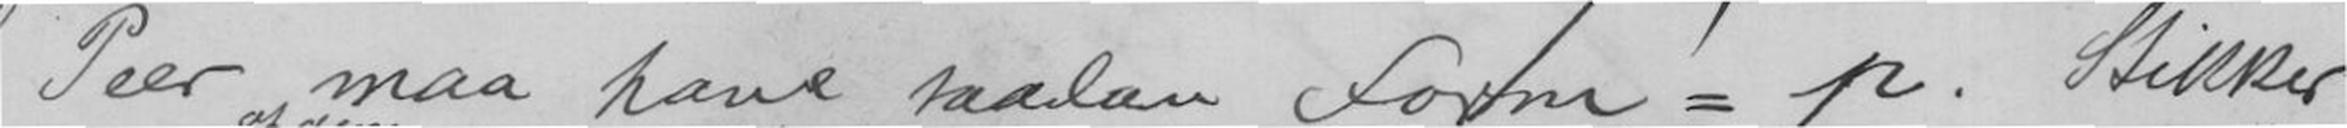Peer maa have saadan form = p. Stikker
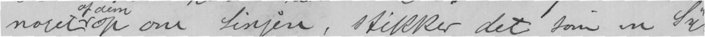noget af dem op om Linjen, stikker det som en Syl
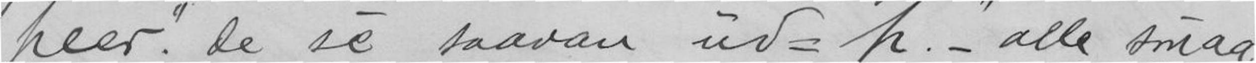peer. De sé saadan ud = p; - alle smaa
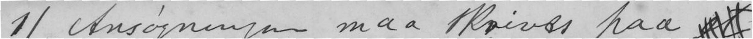1)Ansøgningen maa skrives paa et
